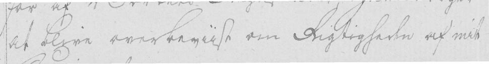at blive overbeviist om Rigtigheden af mit
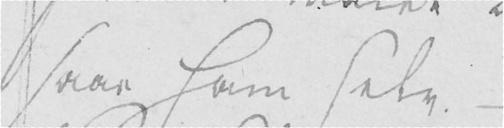saae ham selv. -
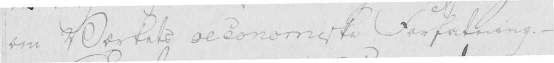om Værkets oeconomiske Forfatning. -
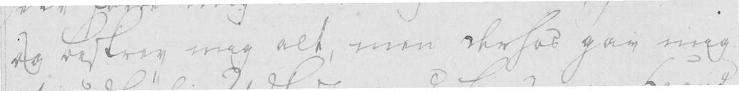og beskrev mig alt, men derhos gav mig
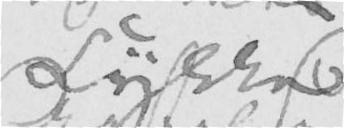Lykke
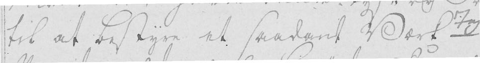til at bestyre et saadant Værk -
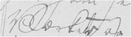Værket og
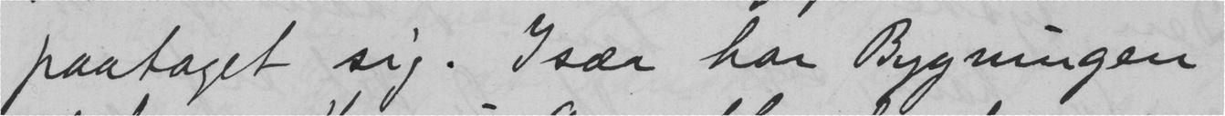paataget sig. Især har Bygningen
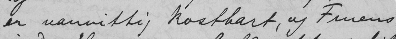er vanvittig kostbart, og Fruens
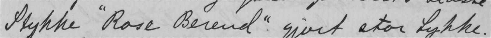Stykke "Rose Berend" gjort stor Lykke.
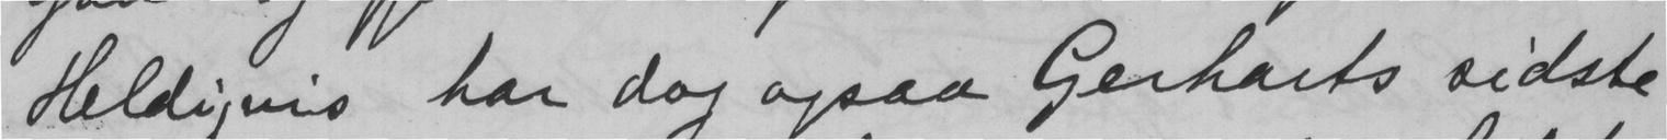Heldigvis har dog ogsaa Gerharts sidste
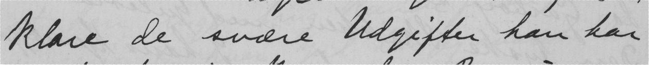klare de svære udgifter han har
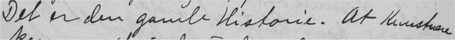Det er den gamle Historie. At Kunstnere
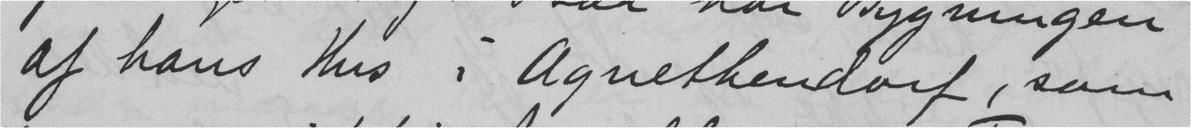af hans Hus i Agnethendorf, som
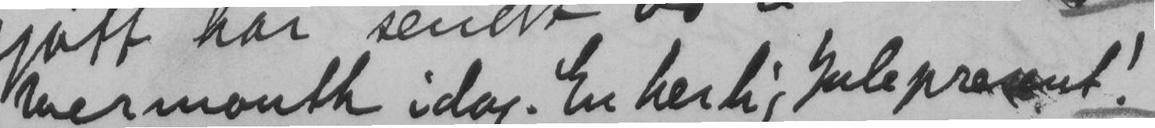wermouth idag. En herlig julepresent!
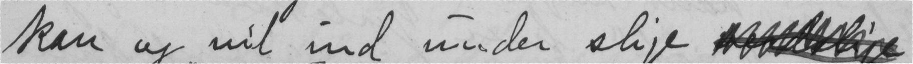kan og vil ind under slige
